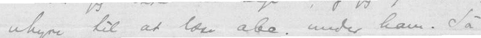uhyre til at læse abc. under ham. Så
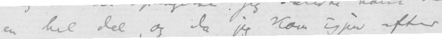en hel del, og da jeg kom igjen efter
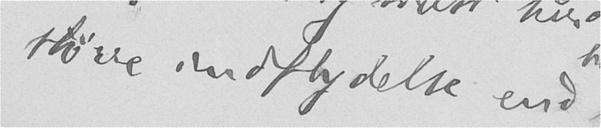større indflydelse end
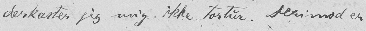derkaster jeg mig ikke tortur. Derimod er
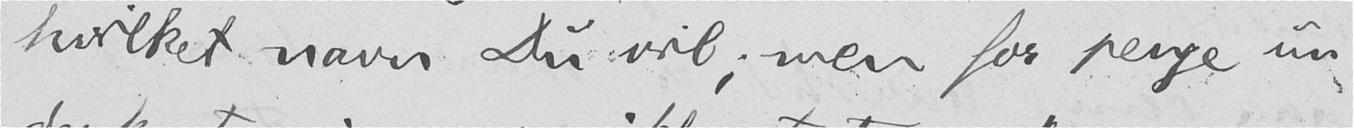hvilket navn Du vil; men for penge un-
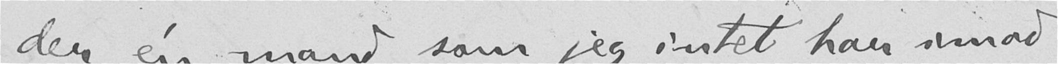der en mand, som jeg intet har imod
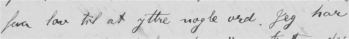faa lov til at yttre nogle ord. Jeg har
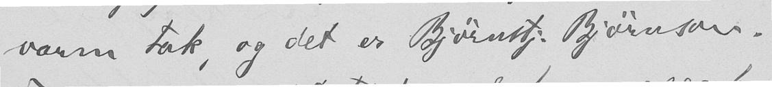varm tak, og det er Bjørnstj. Bjørnson.
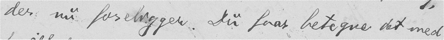der nu foreligger. Du faar betegne det med,
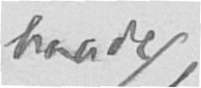baade
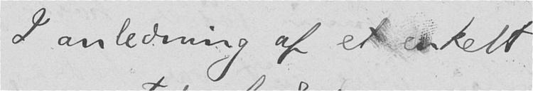I anledning af et enkelt
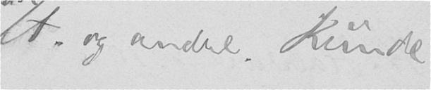A. og andre. Kunde
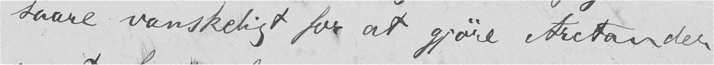saare vanskeligt for at gjøre Arctander
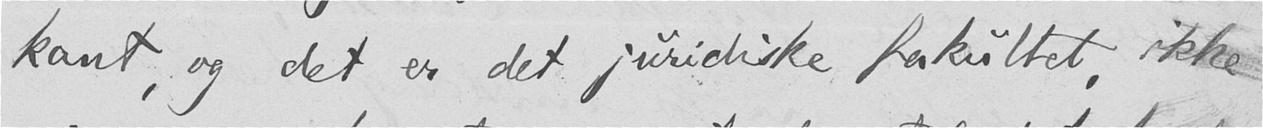kant, og det er det juridiske fakultet, ikke
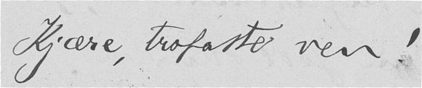Kjære, trofaste ven!
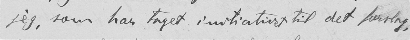jeg, som har taget initiativet til det forslag,
